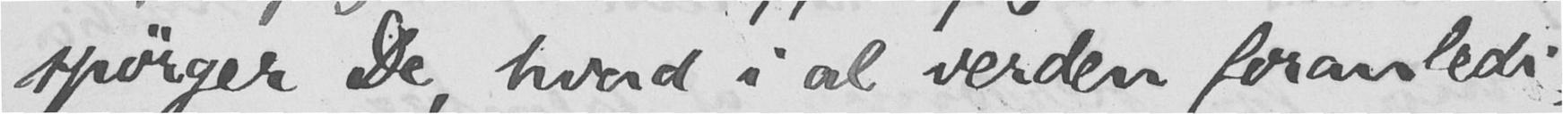spørger De, hvad i al verden foranledi-
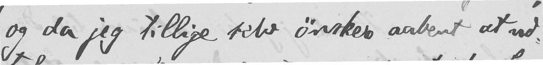og da jeg tillige selv ønsker aabent at ud-
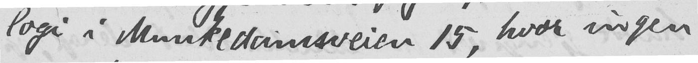logi i Munkedamsveien 15, hvor ingen
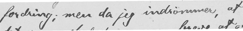fordring; men da jeg indrømmer, at
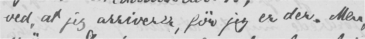ved, at jeg arriverer, før jeg er der. Men,
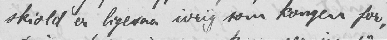skiold er ligesaa ivrig som kongen for,
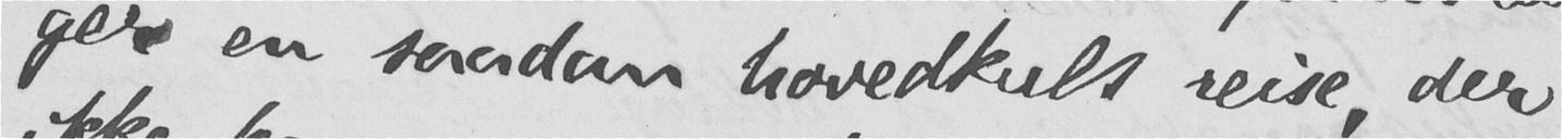ger en saadan hovedkuls reise, der
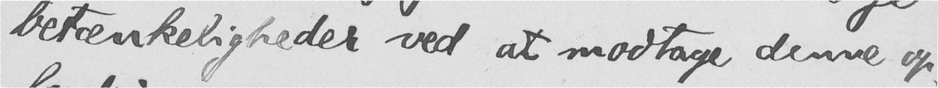betænkeligheder ved at modtage denne op-
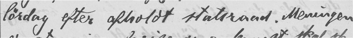lørdag efter afholdt statsraad. Meningen
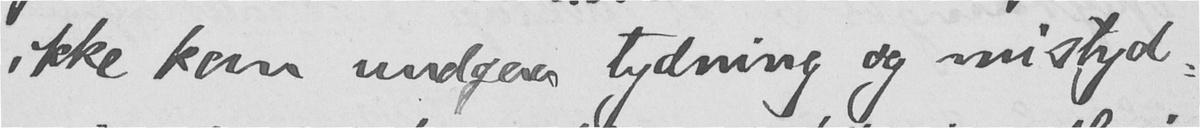ikke kan undgaa tydning og mistyd-
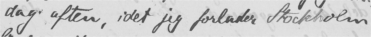dag aften, idet jeg forlader Stockholm
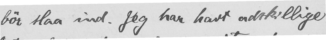bør slaa ind. Jeg har havt adskillige
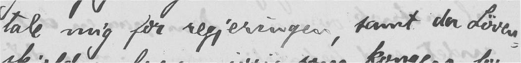tale mig for regjeringen, samt da Løven-
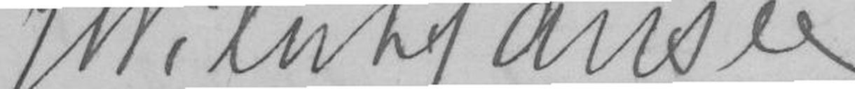Wilhelm Hansen
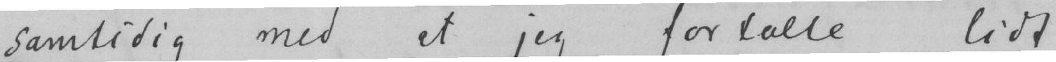samtidig med at jeg fortalte lidt
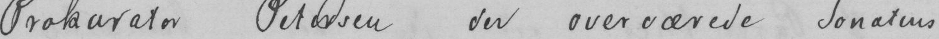Prokarater Petersen, der overværende Sonatens
